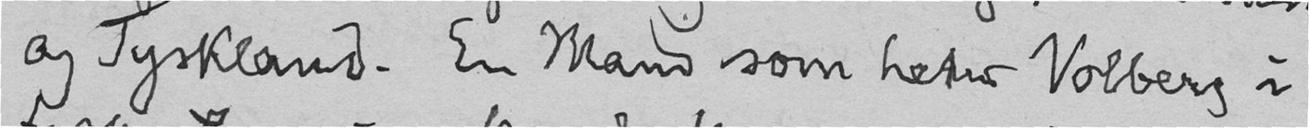og Tyskland. En Mand som heter Volberg i
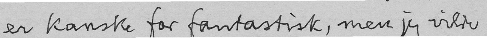er kanske for fantastisk, men jeg vilde
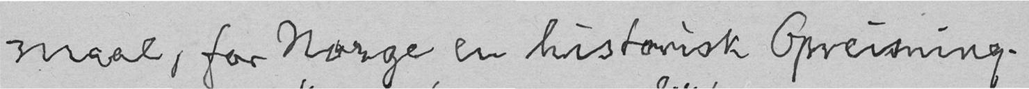maal, for Norge en historisk Opreisning.
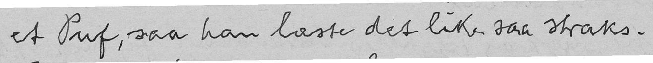et Puf, saa han læste det like saa straks.
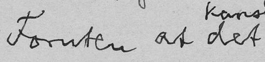Foruten at det
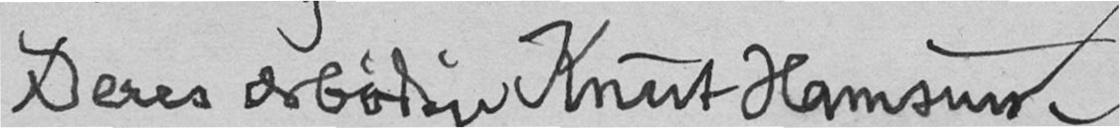Deres ærbødige Knut Hamsun.
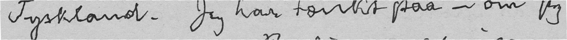Tyskland. Jeg har tænkt paa - om jeg
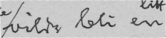vilde bli en
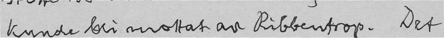kunde bli mottat av Ribbentrop. Det
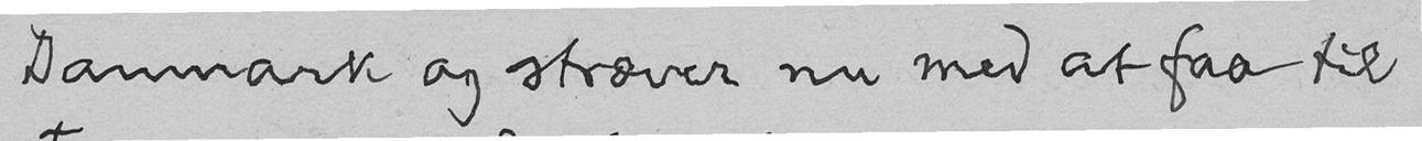Danmark og stræver nu med at faa til
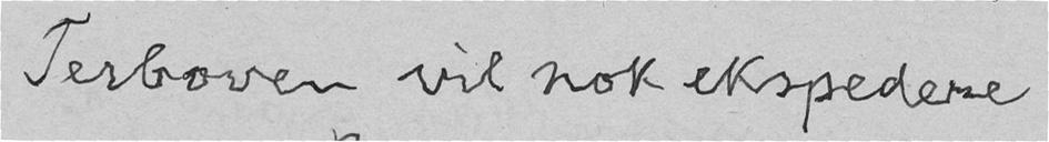Terboven vil nok ekspedere
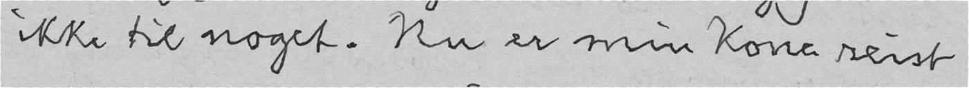ikke til noget. Nu er min Kone reist
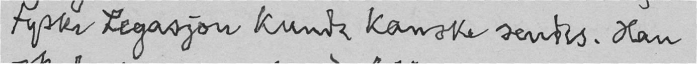tyske Legasjon kunde kanske sendes. Han
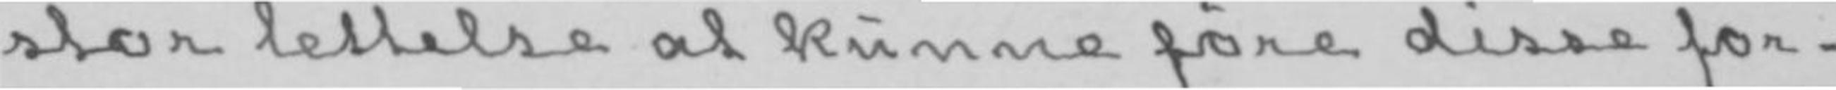stor lettelse at kunne føre disse for-
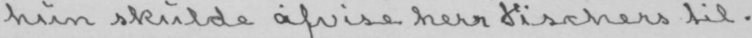hun skulde afvise herr Fischers til-
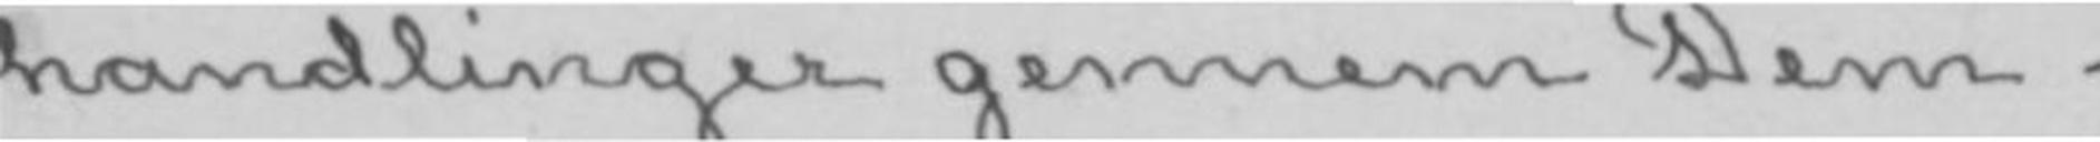handlinger gennem Dem.
